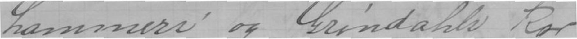Lammers og Grøndahls kor.
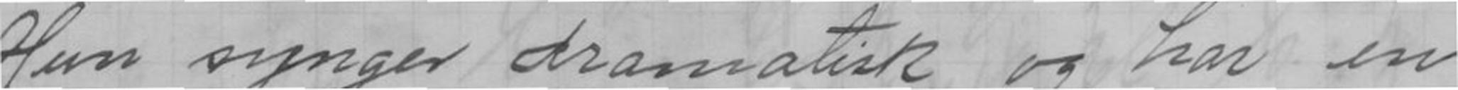Hun synger dramatisk og har en
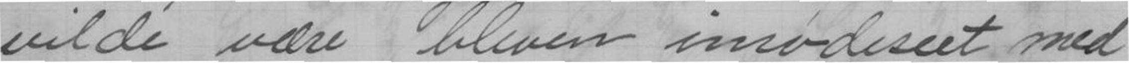vilde være bleven imødeseet med
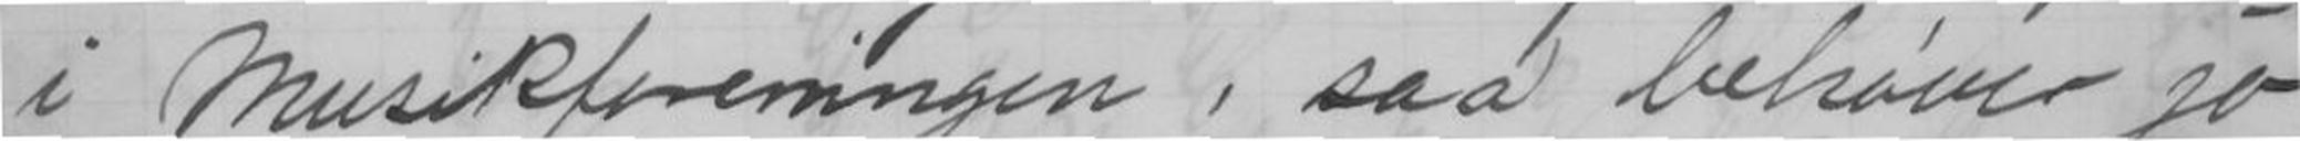i Musikforeningen, saa behøver jo
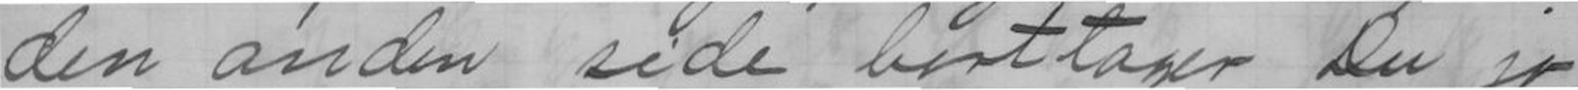den anden side borttager Du jo
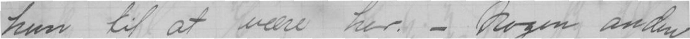hun til at være her. - Nogen anden
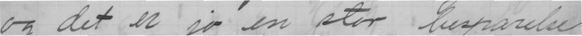og det er jo en stor besparelse
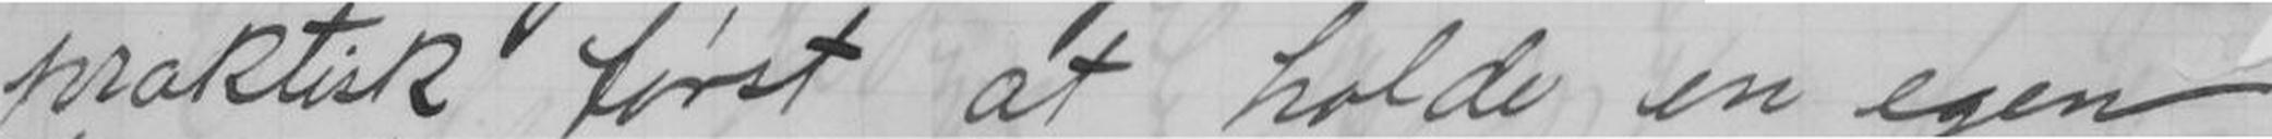praktisk først at holde en egen
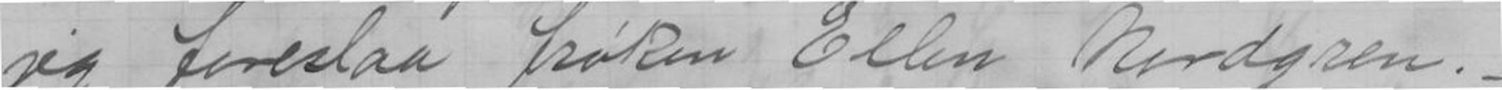jeg foreslaa frøken Ellen Nordgren.
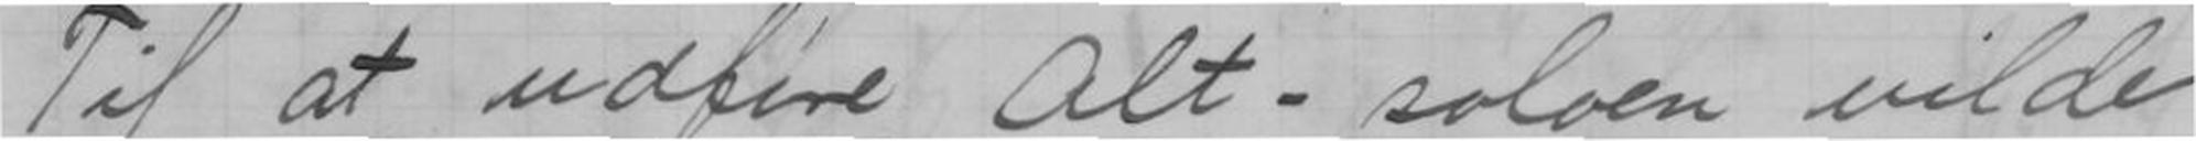Til at udføre Alt-soloen vilde
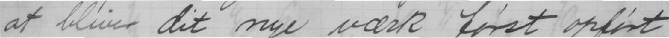at bliver dit nye værk først opført
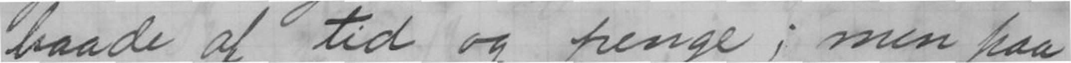baade af tid og penge; men paa
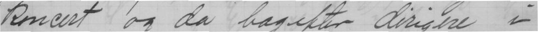koncert og da bagefter dirigere i
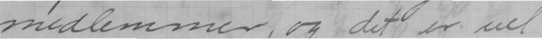medlemmer, og det er vel
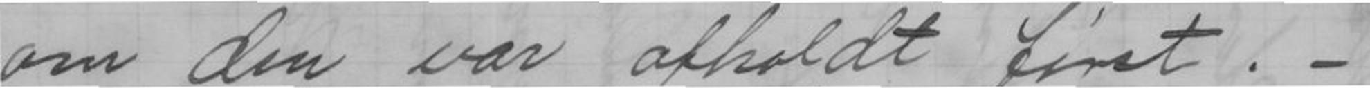om den var afholdt først. -
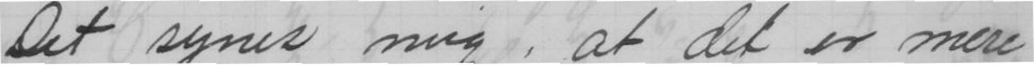Det syner mig, at det er mere
